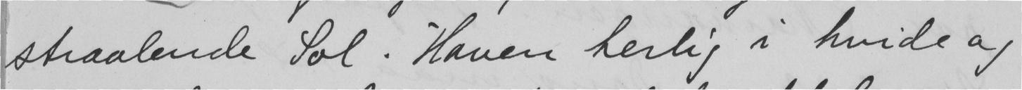straalende Sol. Haven herlig i hvide og
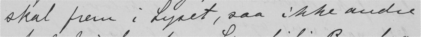skal frem i Lyset, saa ikke andre
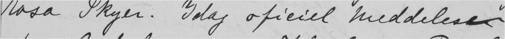rosa Skyer. Idag oficiel meddeleser
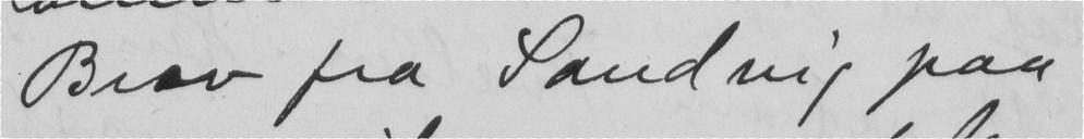Brev fra Sandvig paa
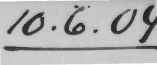10.6.04
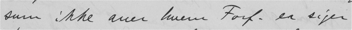som ikke aner hvem Forf. er siger
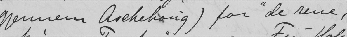gjennem Aschehoug) for "de rene,
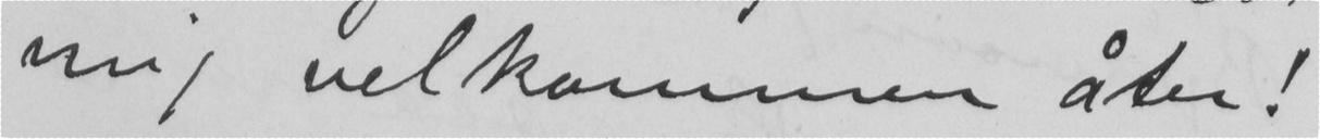mig velkommen åter!
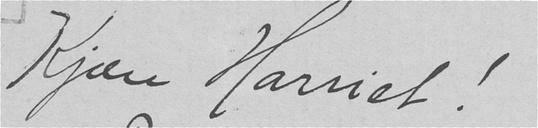Kjære Harriet!
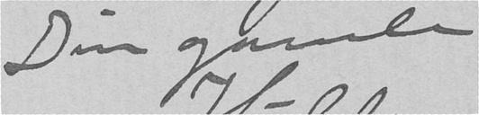Din gamle
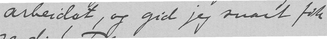arbeidet, og gid jeg snart fik
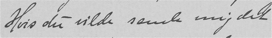Hvis de vilde sende mig det
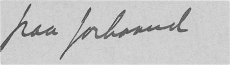paa forhaand
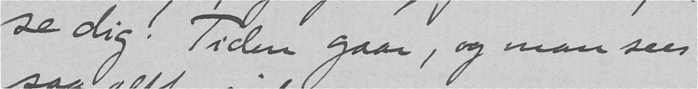se dig! Tiden gaar, og man sees
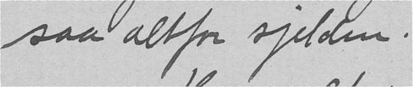saa altfor sjelden.
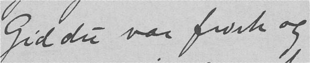Gid du var frisk og
